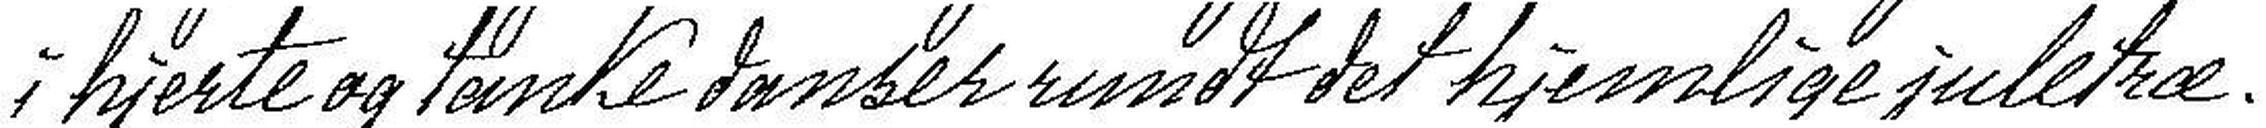i hjerte og tanke danser rundt det hjemlige juletræ.
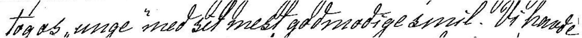tog os unge med sit mest godmodige smil. Vi havde
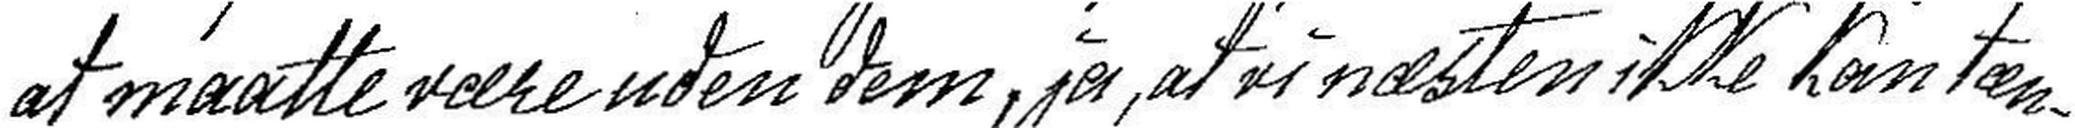at maatte være uden dem, ja, at vi næsten ikke kan tæn-
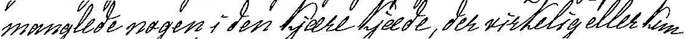mangler nogen i den kjære kjæde, der virkelig eller kun
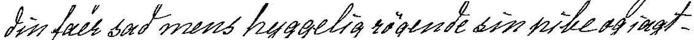din faer sad mens hyggelig røgende sin pibe og iagt-
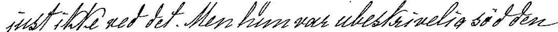just ikke ved det. Men hun var ubeskrivelig sød den
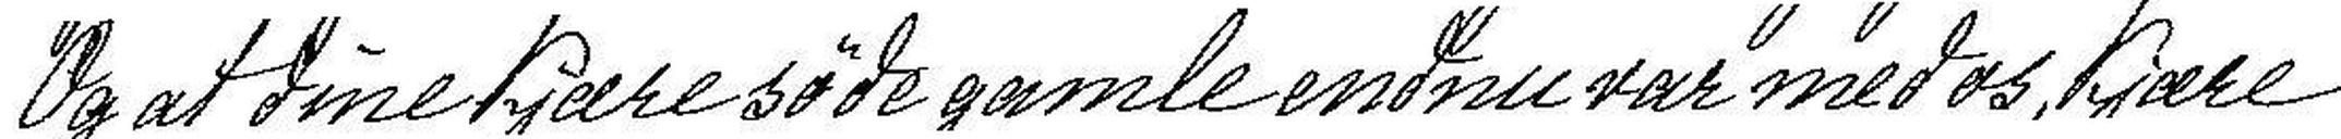Og at dine kjære søde gamle endnu var med os, kjære
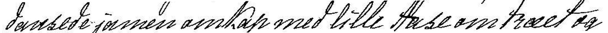dansede jamen omkap med lille Aase om træet og
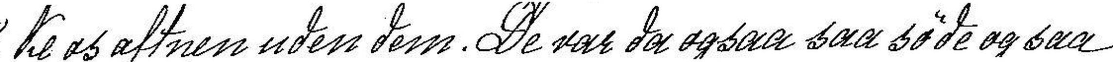ke os aftnen uden dem. De var da ogsaa saa søde og saa
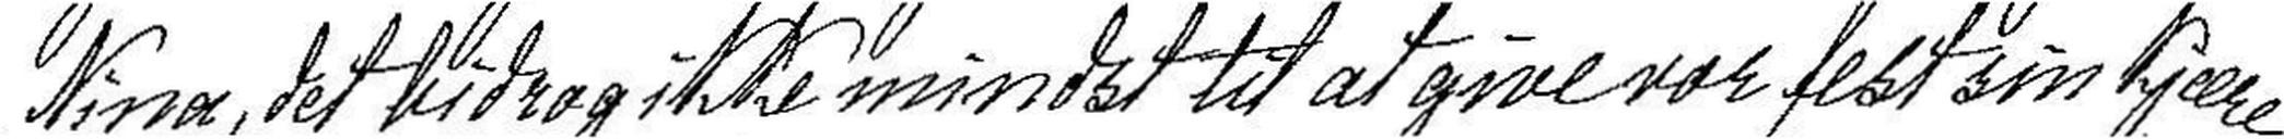Nina, det bidrog ikke mindst til at give vor fest sin kjære
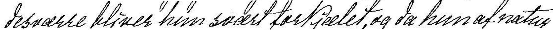desværre bliver hun svært forkjælet, og da hun af natur
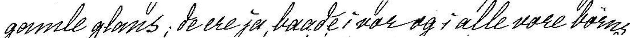gamle glans; de ere ja, baade i vor og i alle vore børns
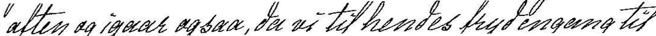aften og igaar ogsaa, da vi til hendes fryd engang til
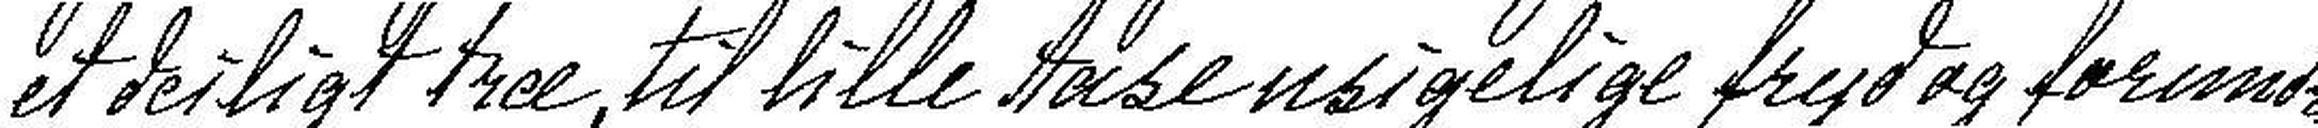et deiligt træ, til lille Aase usigelige fryd og forundring
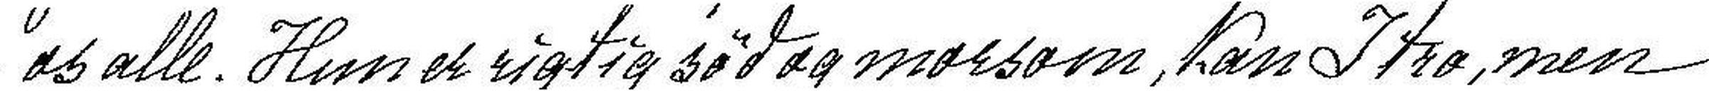os alle. Hun er rigtig sød og morsom, kan I tro, men
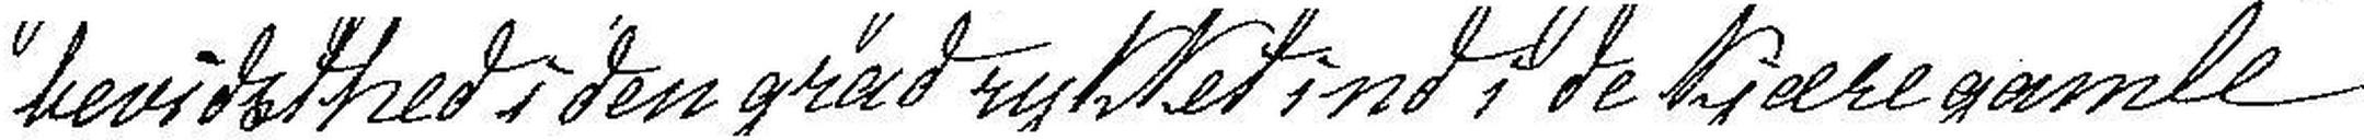bevidshed i den grad rykket ind i de kjære
